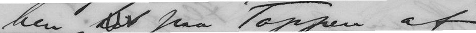hen til paa Toppen af
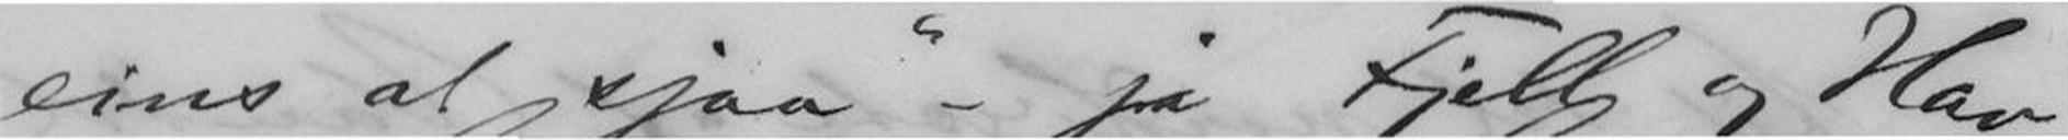eins at sjaa" - ja Fjell og Hav
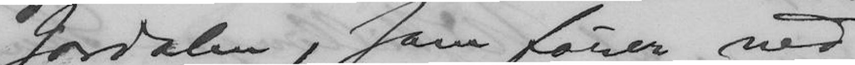Jordalen, som fører ned
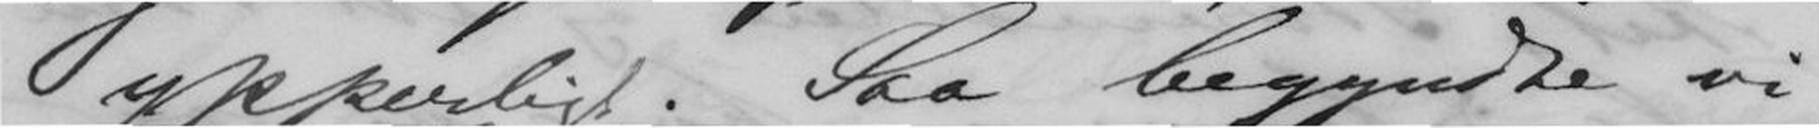ypperligt. Saa begynte vi
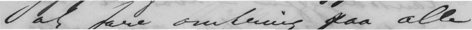at fare omkring paa alle
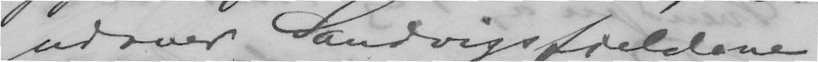udover Sandvigsfjeldene
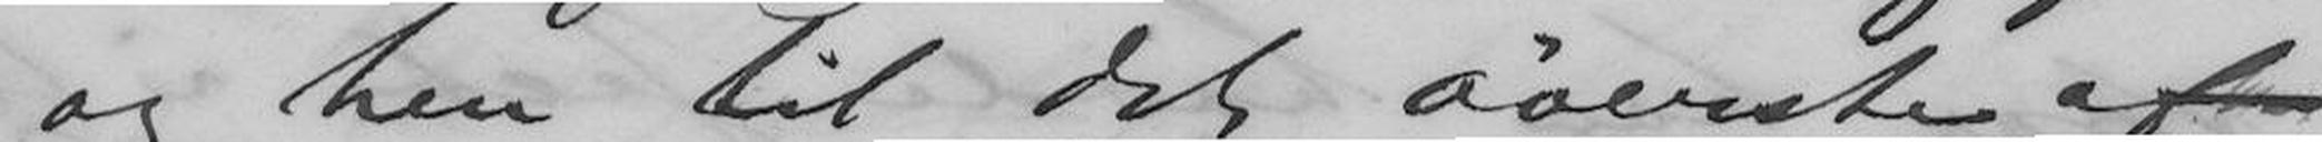og hen til det Øverste af
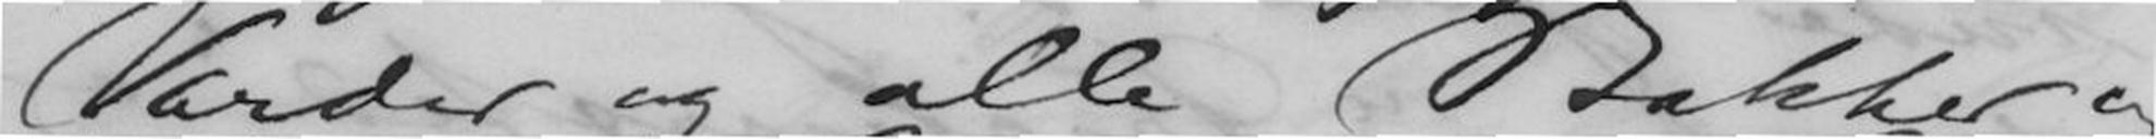Varder og alle Bakker og
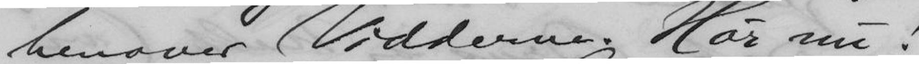henover Vidderne. Hør nu!
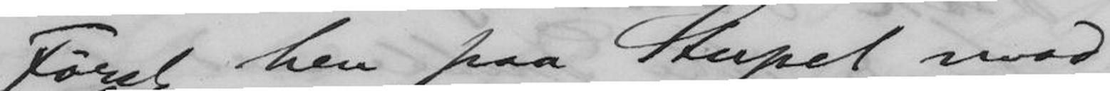Først hen paa Stupet mod
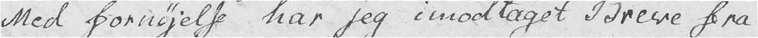Med fornøjelse har jeg imodtaget Breve fra
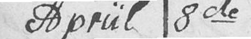Apriil 8de
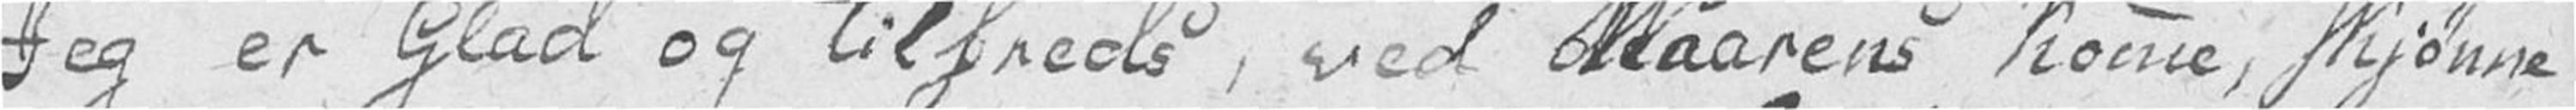Jeg er Glad og tilfreds, ved Vaarens komme, skjønne
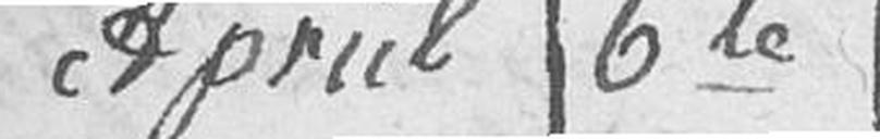Apriil 6te
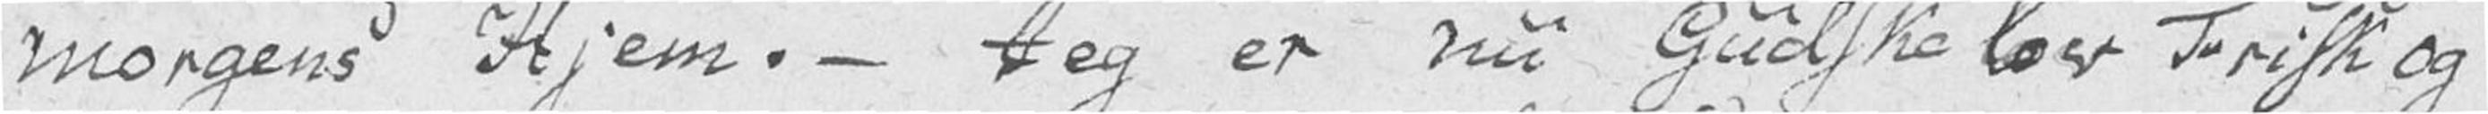morgens Hjem. – Jeg er nu Gudskelov Frisk og
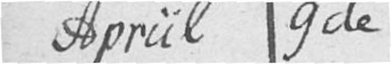Apriil 9de
